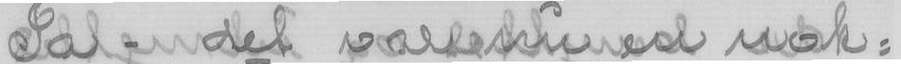Ja - det var nu en nok-
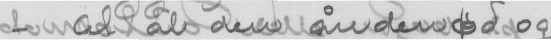- At al den åndenød og
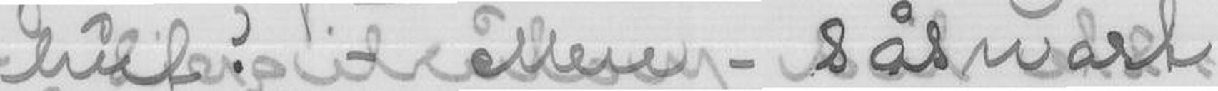huf! - Men - såsnart
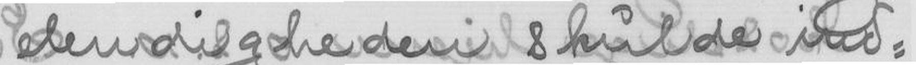elendighederi skulde ind-
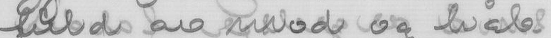fuld av mod og håb
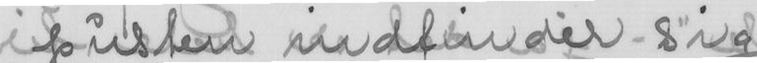pusten indfinder sig
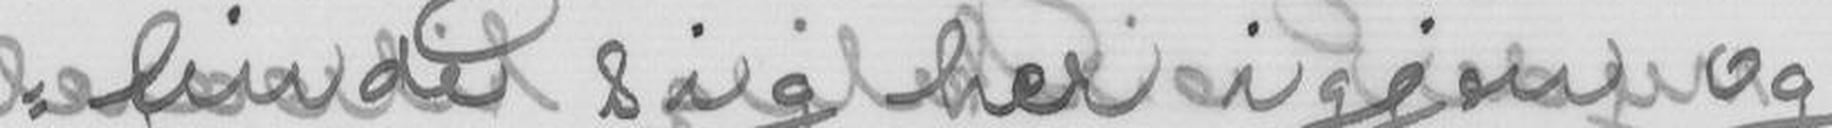finde sig her igjen og
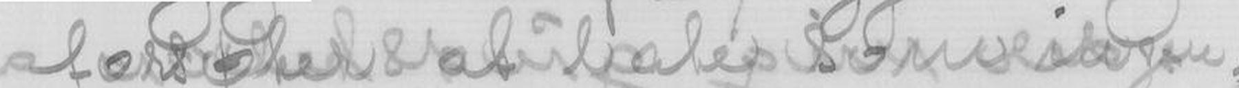forsøker at late som ingen-
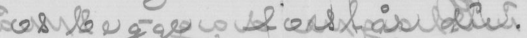os begge, forstår du.
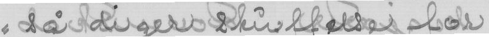så diger skuffelse for
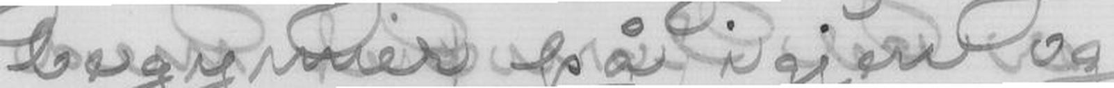begynner på igjen og
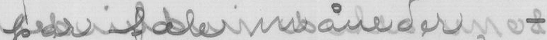par fæle måneder, -
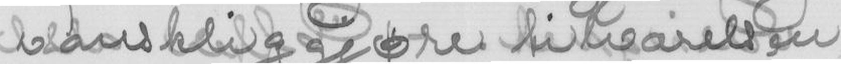vanskliggjøre tilværelsen
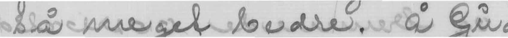så meget bedre. Å Gud,
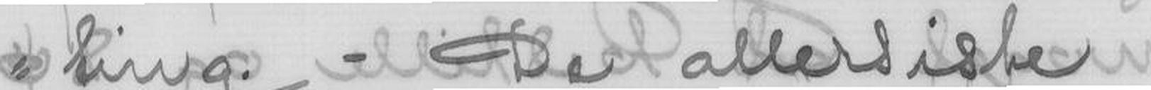ting. - De allersiste
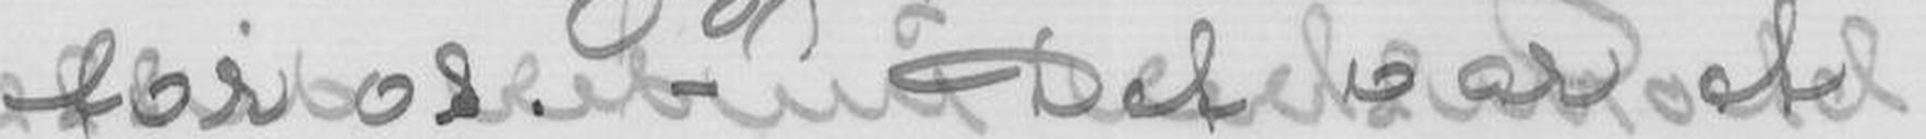for os. - Det var et
